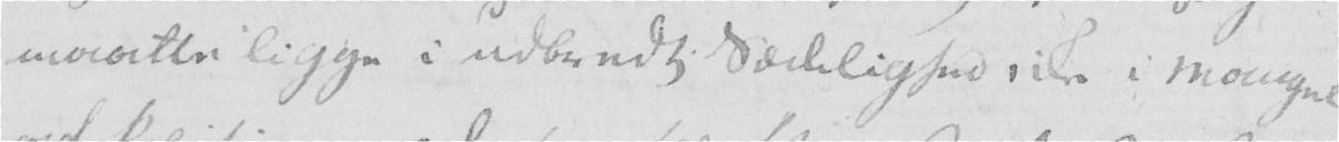maatte ligge i udbrudt Sædelighed, ikke i mangel
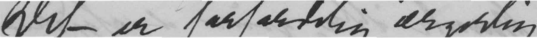Det er forfærdelig ærgerlig
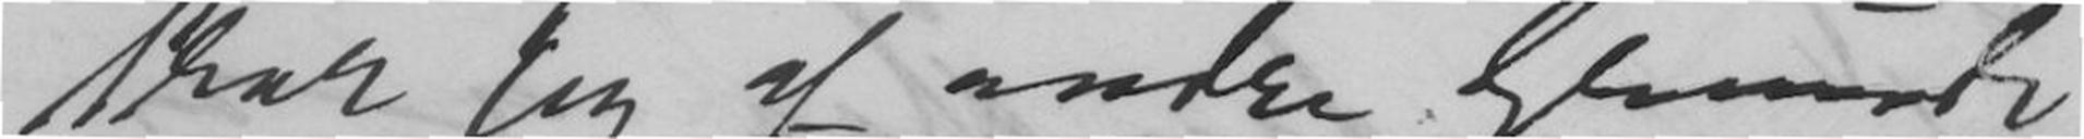tror jeg af andre Grunde
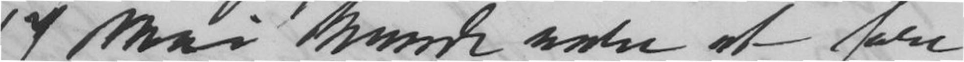17 Mai kunde være at fore-
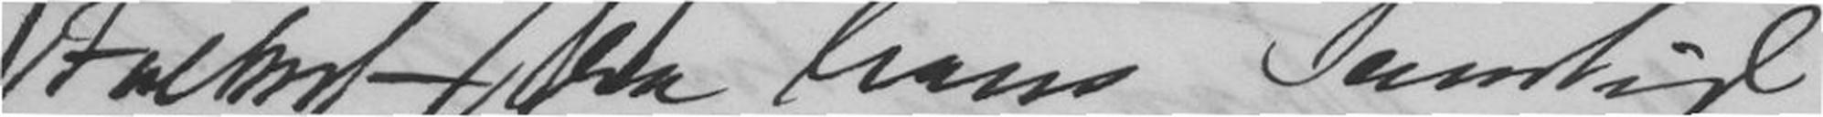Folket fra hans Samtid.
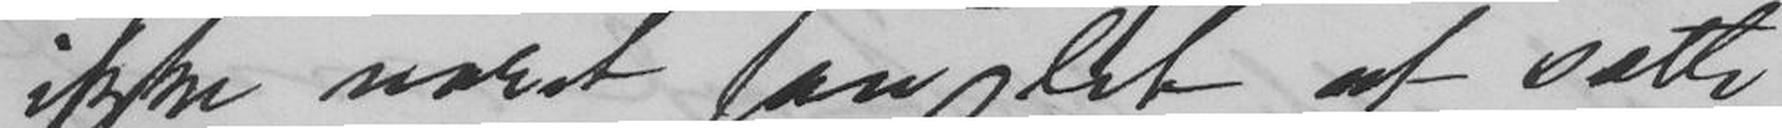ikke været saa let at sætte
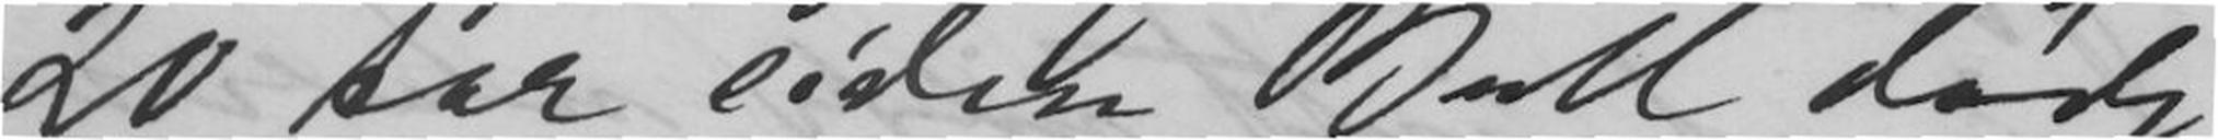20 Aar siden Bull døde
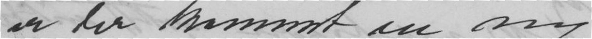er der kommet en ny
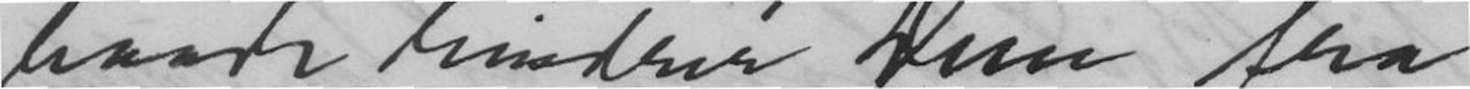baade hindrer Dem fra
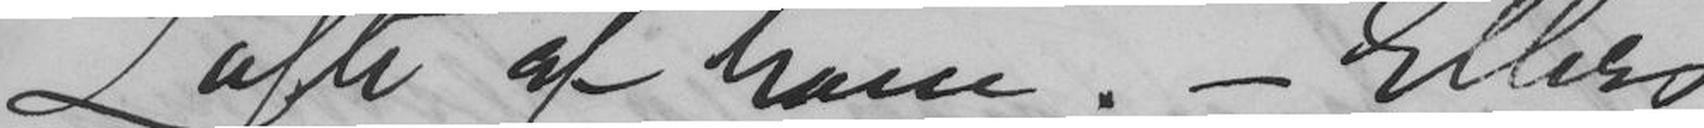Løfte af ham. - Ellers
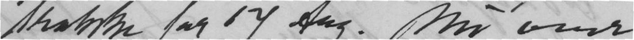trække for 17 Aug. Nu over
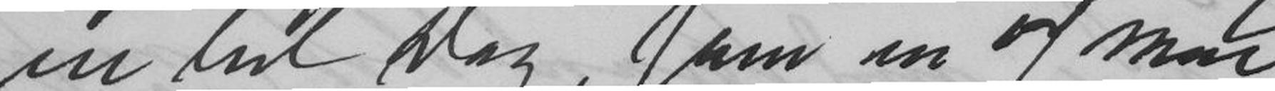en hel Dag, som en 17 Mai.
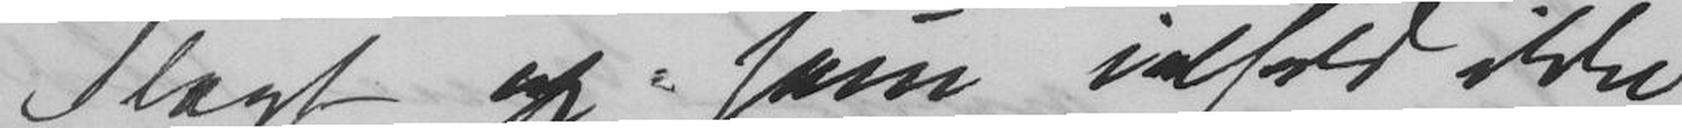Slægt op som ialfald ikke
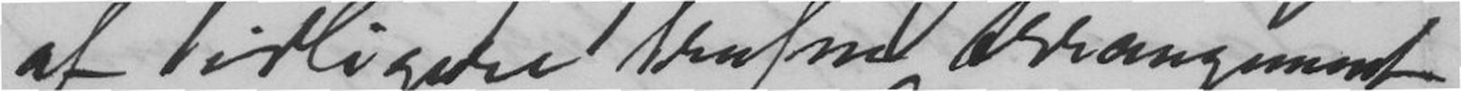at tidligere trufne Arrangement
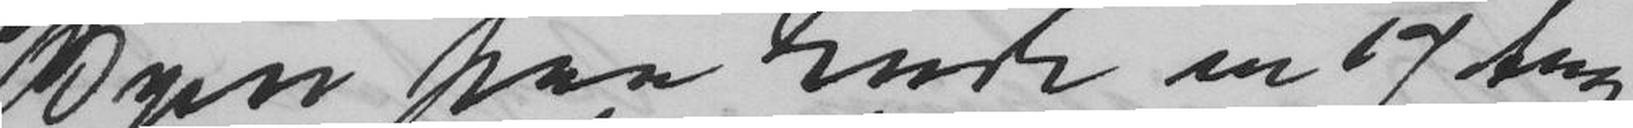Byen paa Ende en 17 Aug.
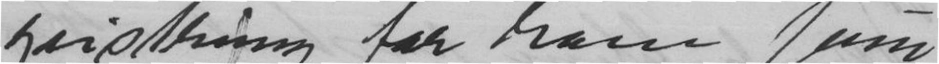geistring for ham som
